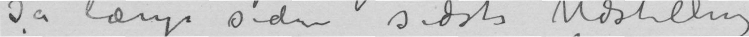så længe siden sidste Udstilling
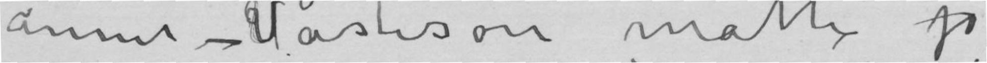og annet – Vasteson måtte jo
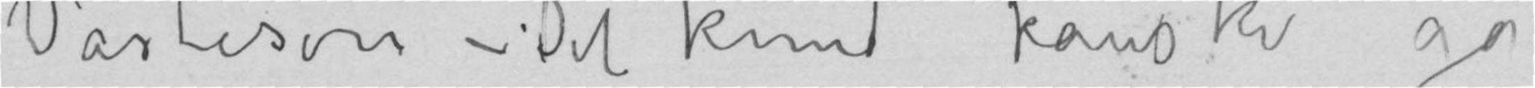Vasteson – Det kunde kanske gå
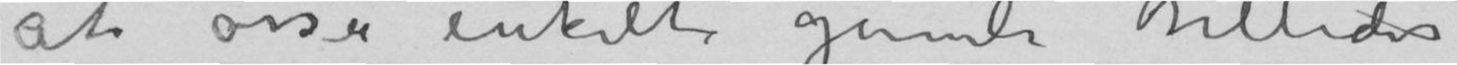at osså enkelte gamle Billeder
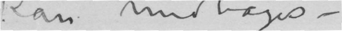kan medtages –
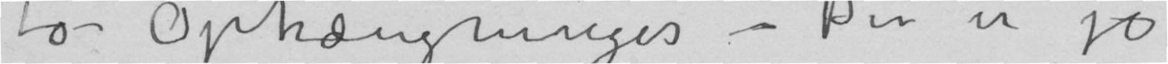to Ophængninger – Der er jo
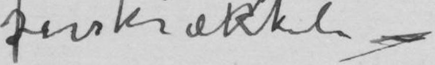forskrækkeli –
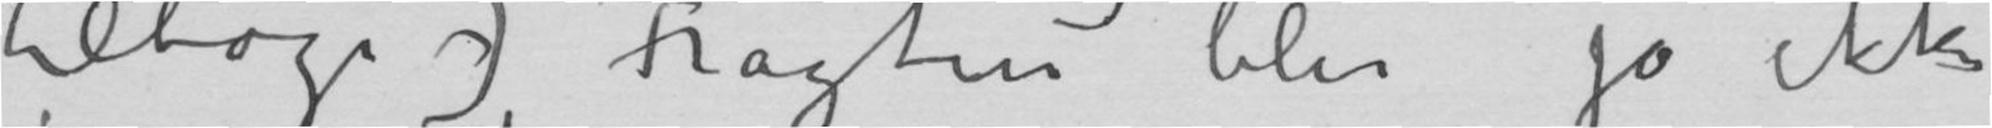tilbage –) Fragten blir jo ikke
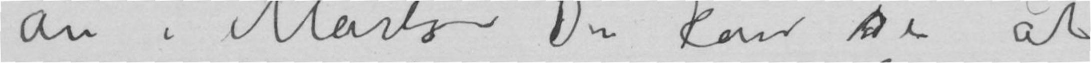an i MartsDu kan si at
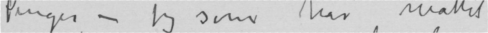Penger – jeg som har måttet
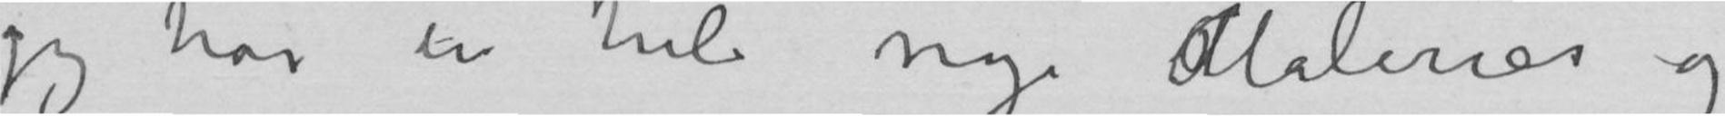jeg har en hel nye Malerier og
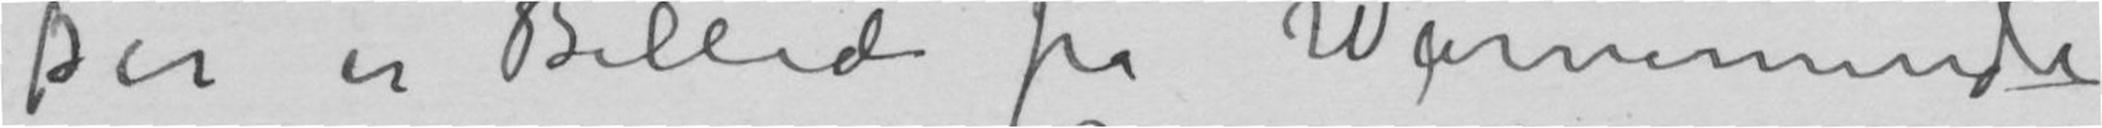Der er Billede fra Warnemünde
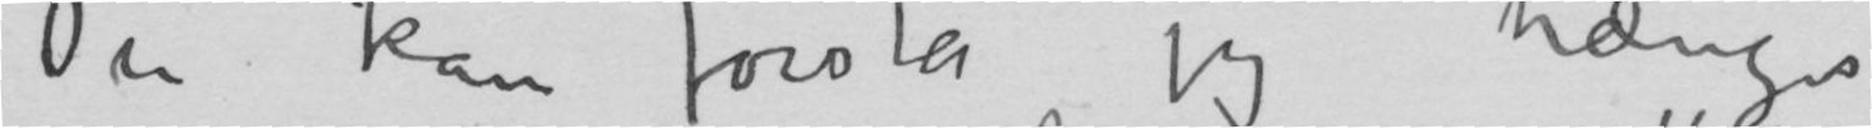Du kan jo forstå jeg trænger
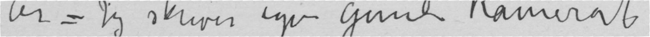År – Jeg skriver igjen gamle Kamerat
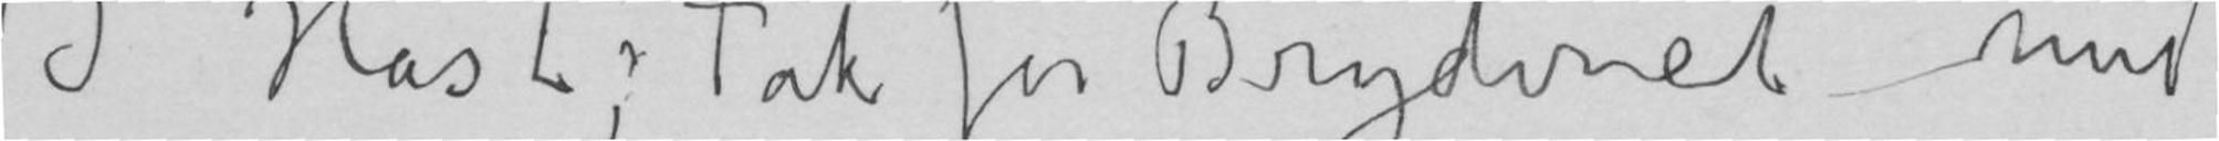I Hast; Tak for Bryderiet med
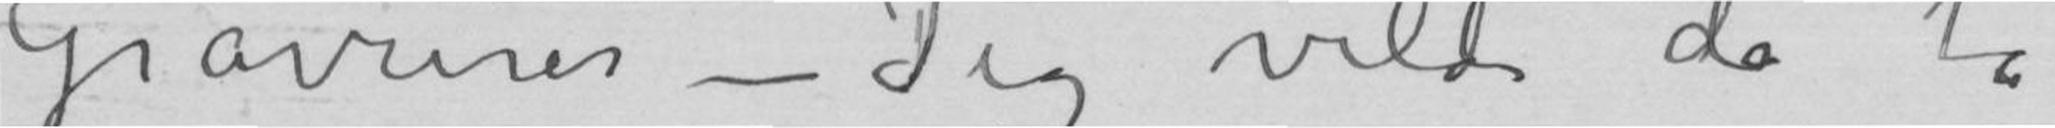Gravurer – Jeg vilde da ta
